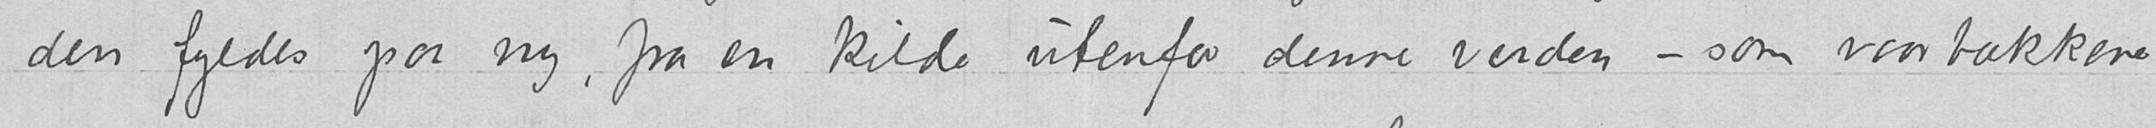den fyldes paa ny, fra en kilde utenfor denne verden - som vaarbækkene
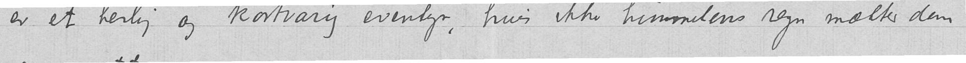er et herlig og kortvarig eventyr, hvis ikke himmelens regn mætter dem
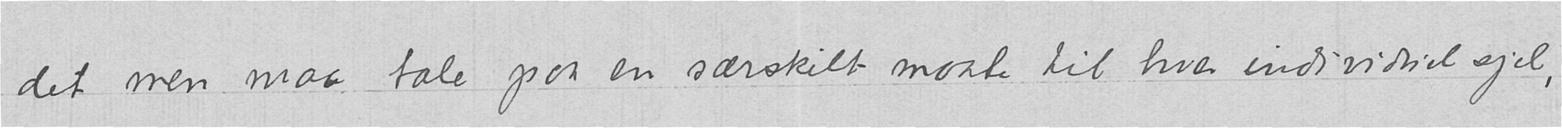det men maa tale paa en særskilt maate til hver individuel sjel,
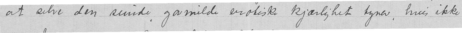at selve den sunde, gavmilde erotiske kjærlighet tyner, hvis ikke
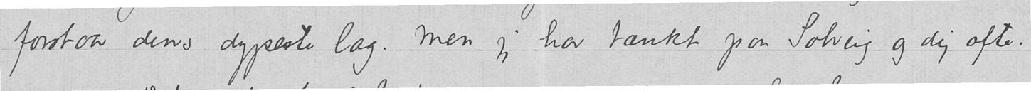forstaar dens dypeste lag. Men jeg har tænkt paa Solveig og dig ofte.
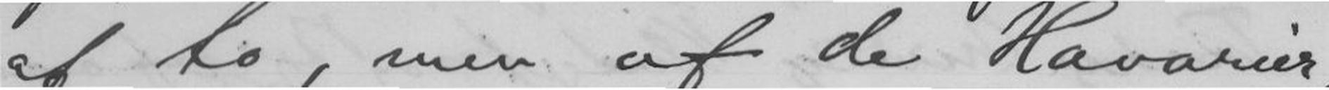af to, men af de Havarier,
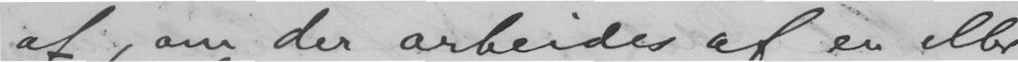af, om der arbeides af en eller
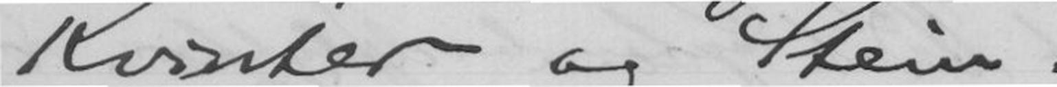Kvister og Stein.
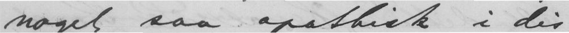noget saa apathisk i dis-
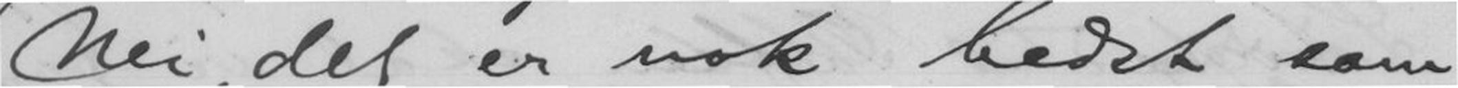Nei, det er nok bedst som
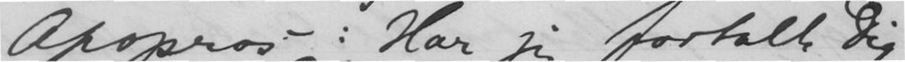Apopros: Har jeg fortalt Dig
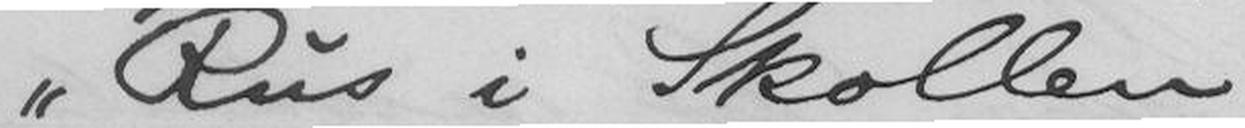" Rus i Skollen,
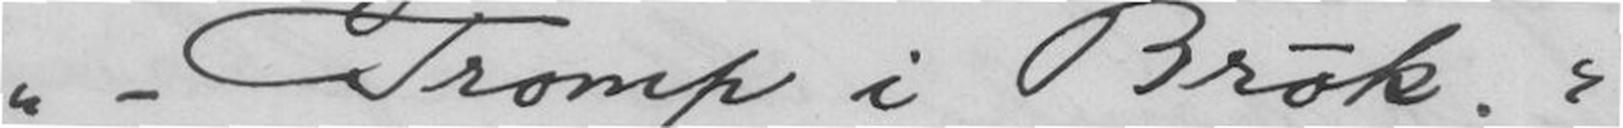" - Tromp i Brok."
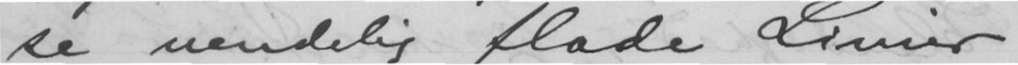se uendelig flade Linier,
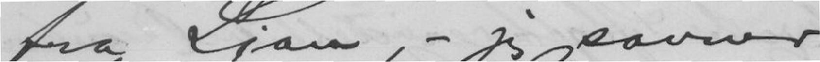fra Ljan, - jeg savner
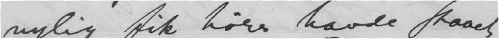jeg nylig fik høre havde staaet
